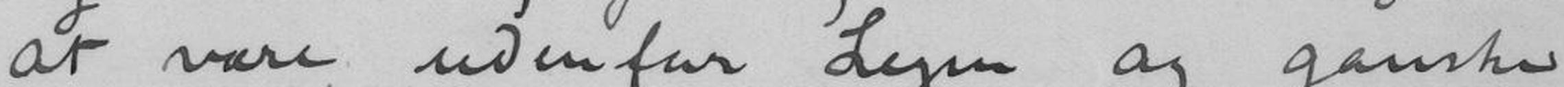at være udenfor Legen og ganske
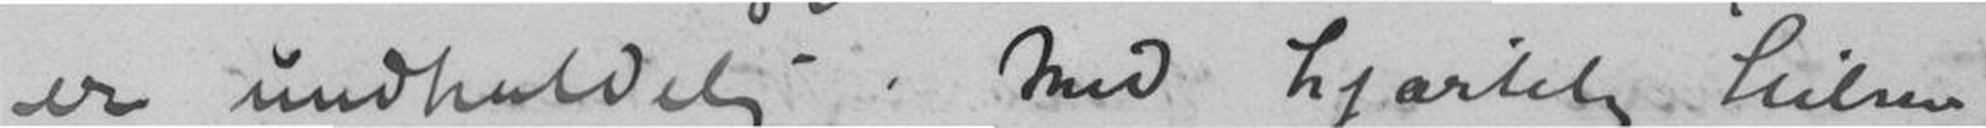er uudholdelig. Med hjærteleg hilsen
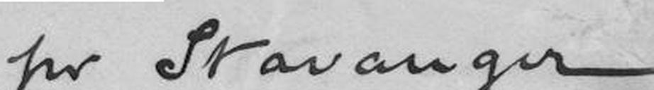pr. Stavanger
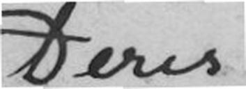Deres
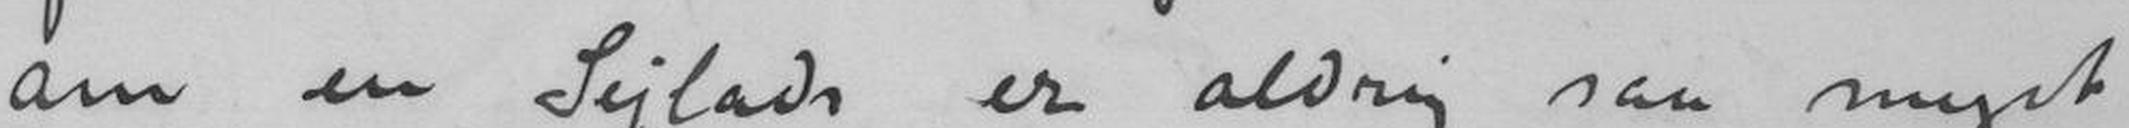om en Sejlade er aldrig saa meget
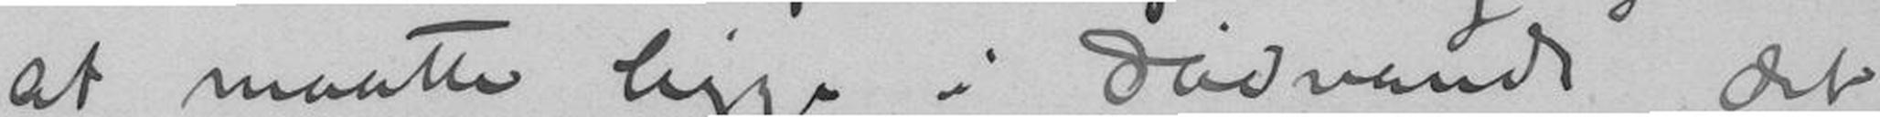at maatte ligge i Dødvandet, det
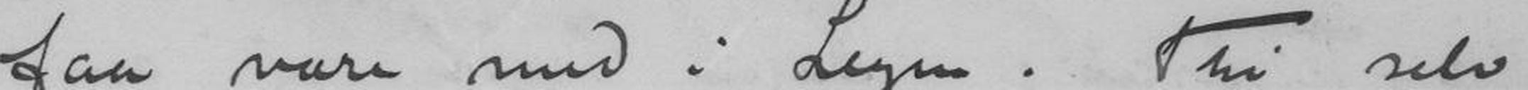faa vare med i Legen. Thi selv
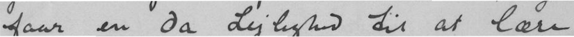faar en da Lejleghed til at lære
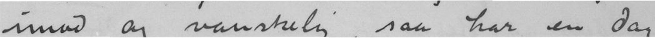imod og vanskelig, saa har en dog
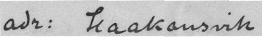adr. Haakonsvik
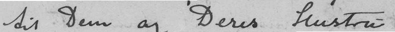til Dem og Deres Hustru
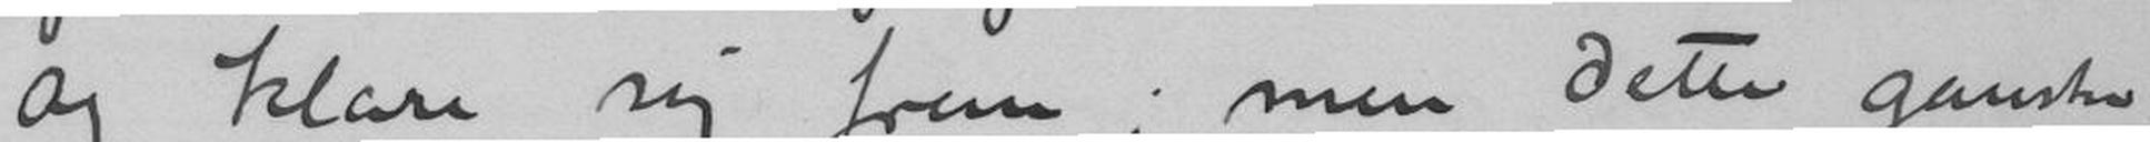og klare sig frem; men dette ganske
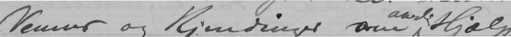Venner og Kjendinger om Hjælp
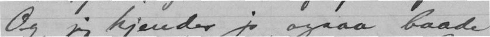Og jeg kjender jo ogsaa baade
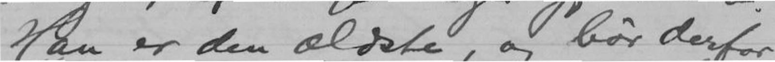Han er den ældste, og bør derfor
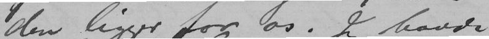den ligger for os. Jeg havde
